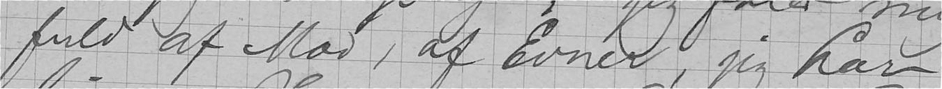fuld af Mod, af Evner, jeg har
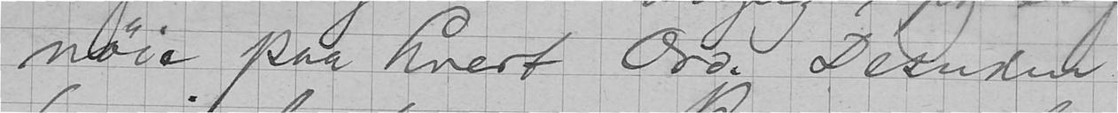nøie paa hvert Ord. Desuden
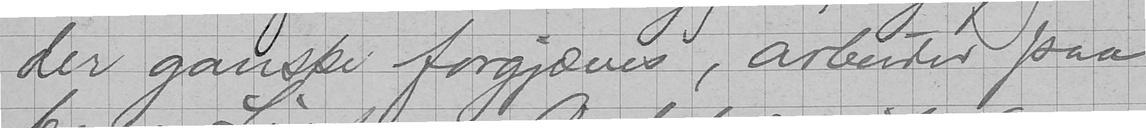der ganske forgjæves, arbeider paa
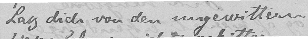Lass dich von ungewittern
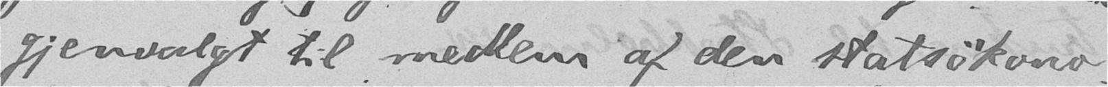gjenvalgt til medlem af den statsøkono-
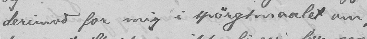derimod for mig i spørgsmaalet om,
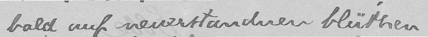bald auf neuerstandnen blüthen
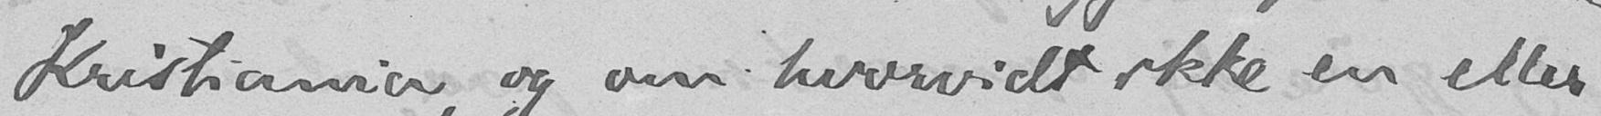Kristiania, og om hvorvidt ikke en eller
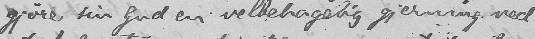gjøre sin Gud en velbehagelig gjerning ved
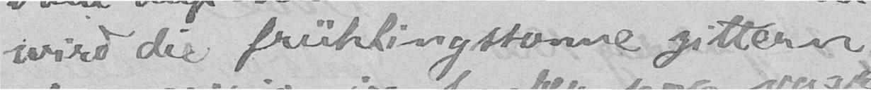wird die frühlingssonne zittern.
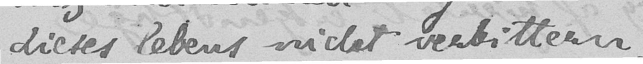dieses lebens nicht verbittern,
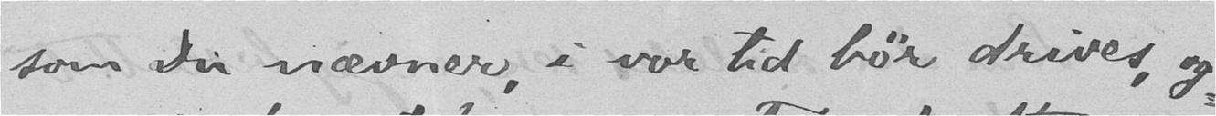som Du nævner, i vor tid bør drives, og-
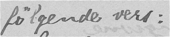følgende vers:
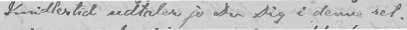Imidlertid udtaler jo Du Dig i denne ret-
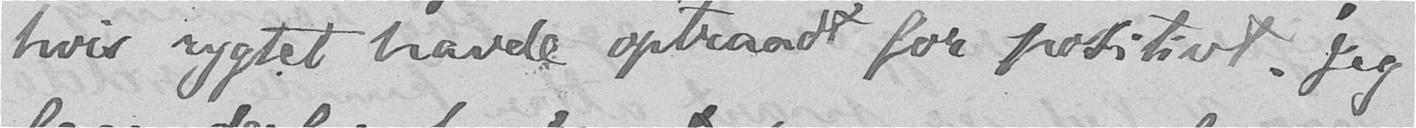hvis rygtet havde optraadt for positivt. Jeg
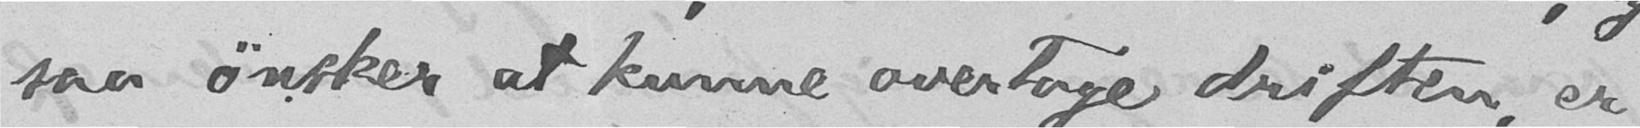saa ønsker at kunne overtage driften, er
